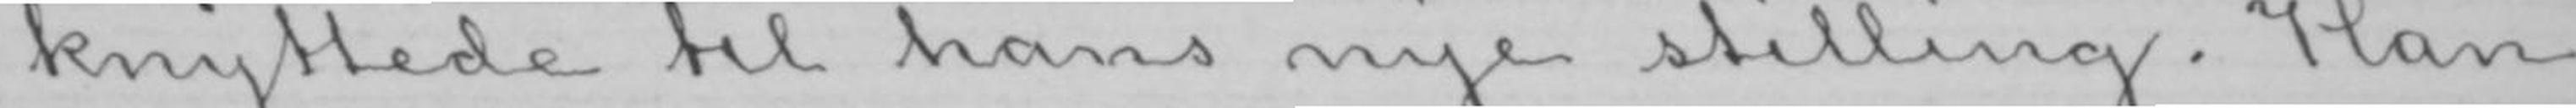knyttede til hans nye stilling. Han
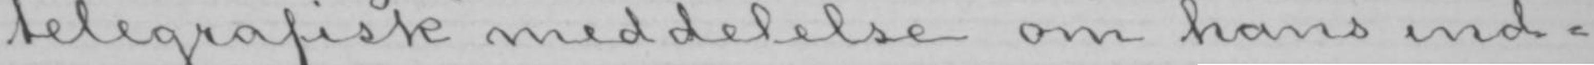telegrafisk meddelelse om hans ind-
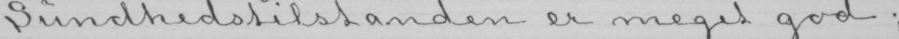Sundhedstilstanden er meget god;
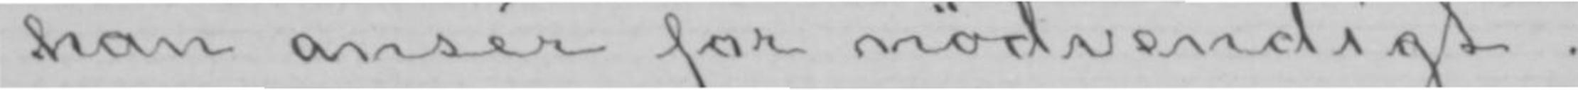han ansér for nødvendigt.
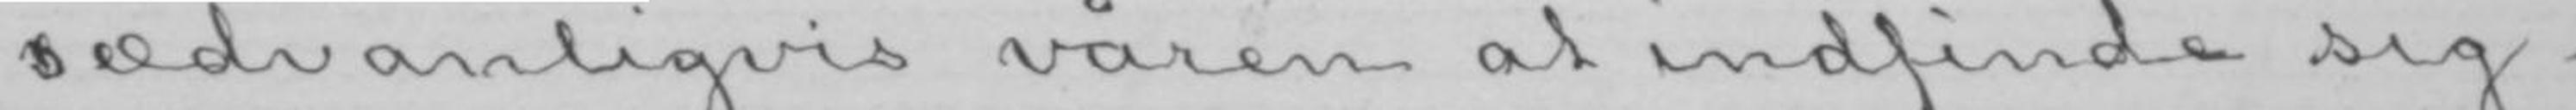sædvanligvis våren at indfinde sig.
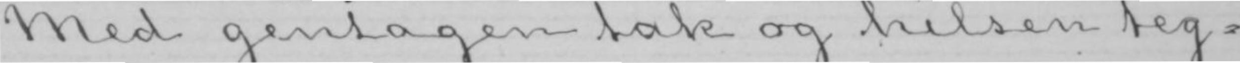Med gentagen tak og hilsen teg-
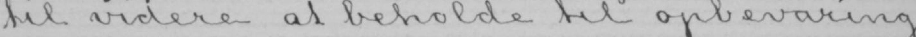til videre at beholde til opbevaring
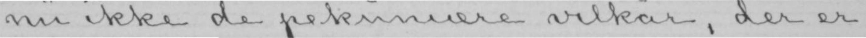nu ikke de pekuniære vilkår, der er
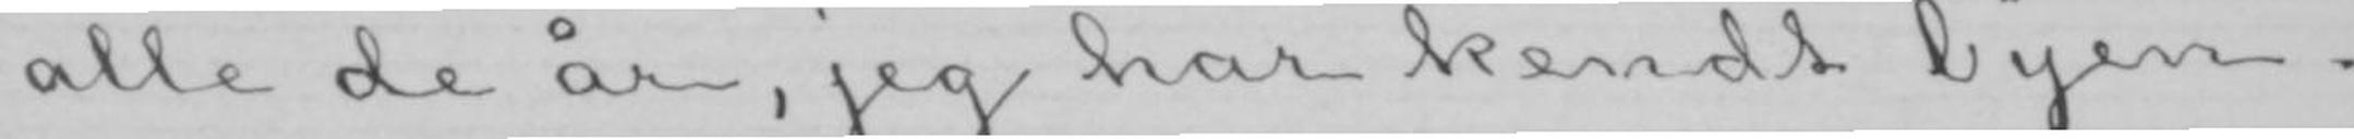alle de år, jeg har kendt byen.
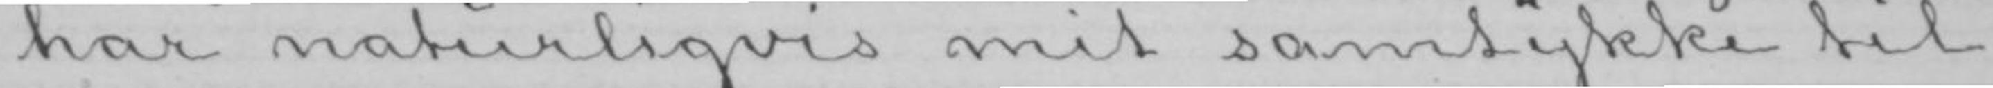har naturligvis mit samtykke til
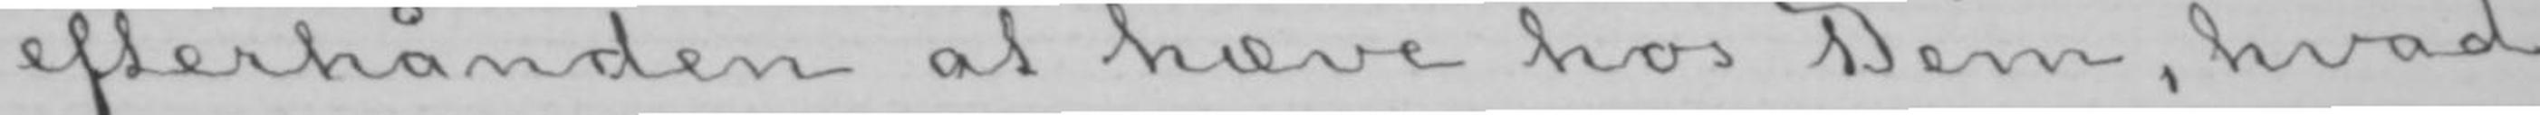efterhånden at hæve hos Dem, hvad
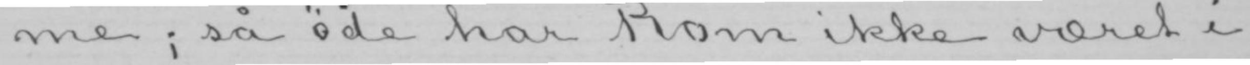me; så øde har Rom ikke været i
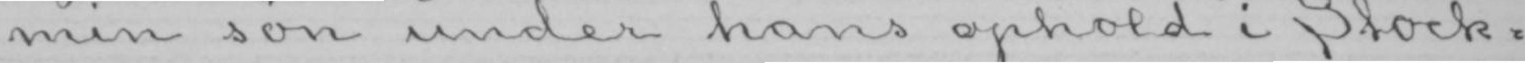min søn under hans ophold i Stock-
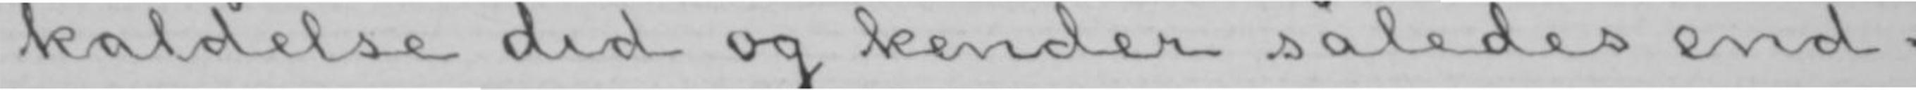kaldelse did og kender således end-
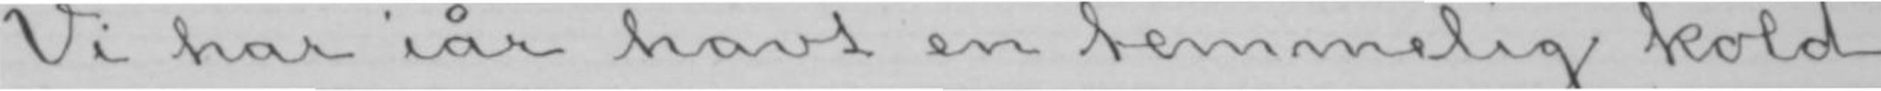Vi har iår havt en temmelig kold
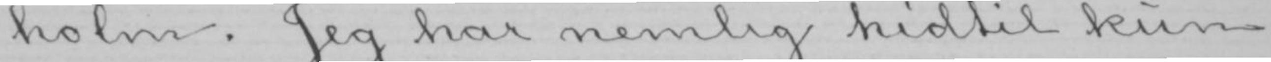holm. Jeg har nemlig hidtil kun
